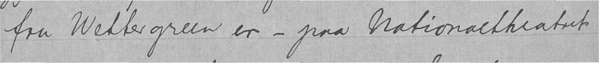fru Wettergreen er - paa Nationaltheatret
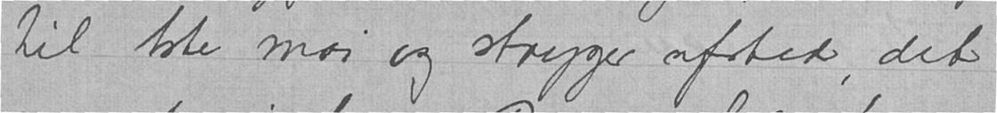til 1ste mai og stryger afsted, det
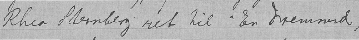Rhea Sternberg ret til «En fremmed»,
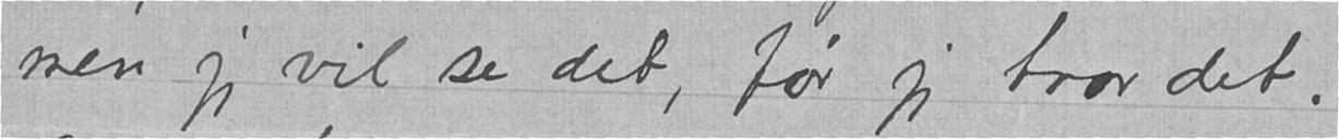men jeg vil se det, før jeg tror det.
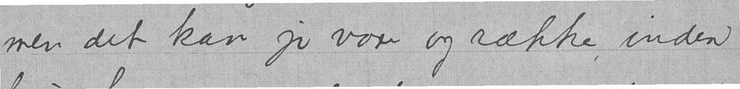men det kan jo vare og række, inden
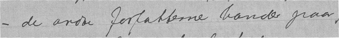- de andre forfatterne bander paa,
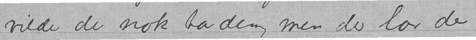vilde de nok ta den, men der lar de
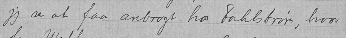jeg se at faa anbragt hos Fahlstrøm, hvor
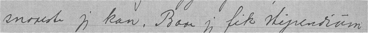snareste jeg kan. Bare jeg fik stipendium
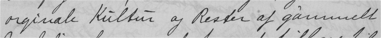orginale Kultur og Resser af gammelt
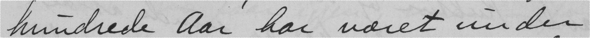hundrede aar har været under
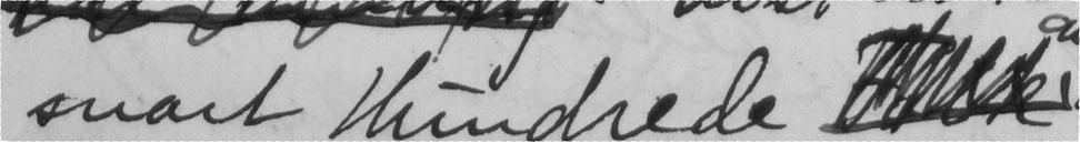snart Hundrede
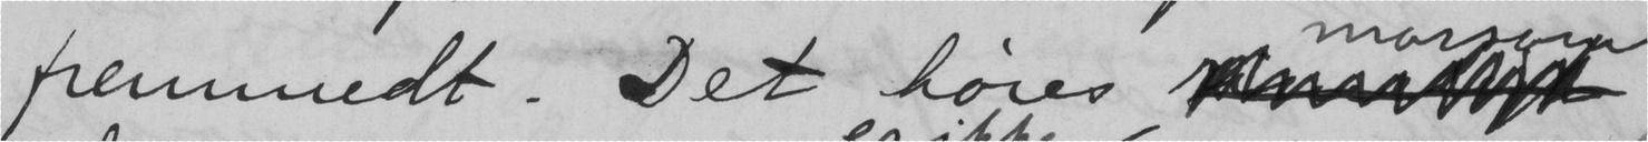fremmedt. Det høres
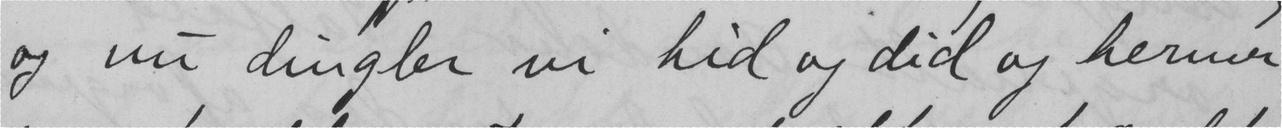og nu dingler vi hid og did og hermer
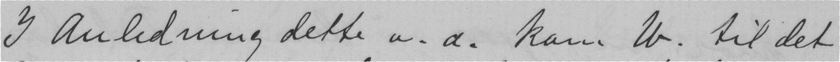I Anledning dette v. a. kom W. til det
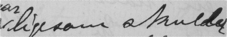ligesom skulde
